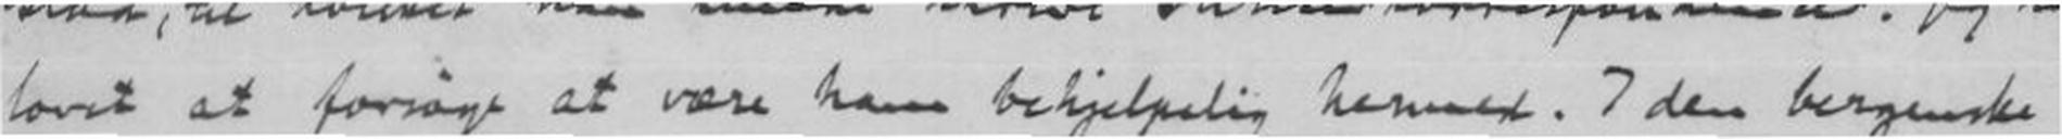lovet at forsøge at være ham behjelpelig hermed. I den bergenske
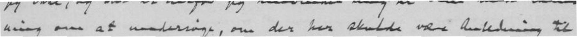ning om at undersøge, om der her skulde være Anledning til
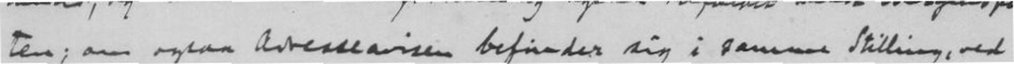ten; om ogsaa Adresseavisen befinder sig i samme Stilling, ved
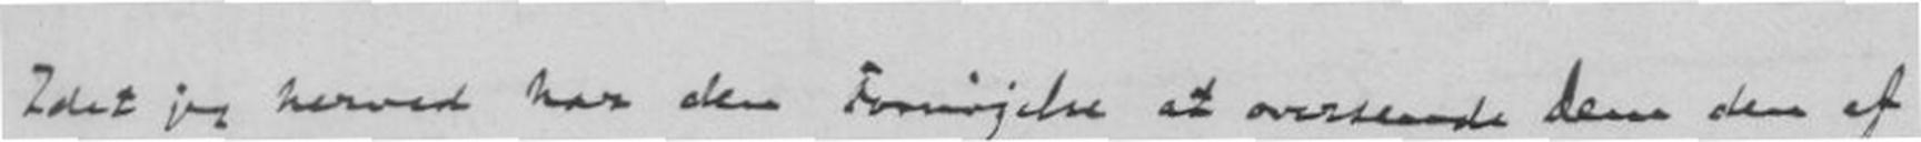Idet jeg herved Har den Fornøjelse at oversende Dem den af
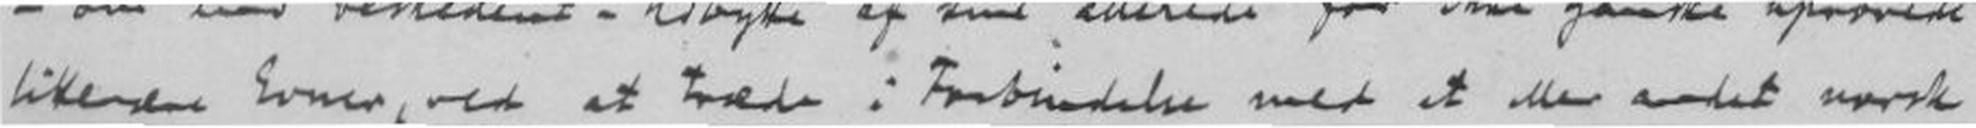litterære Evner, ved at træde i Forbindelse med et eller andet norsk
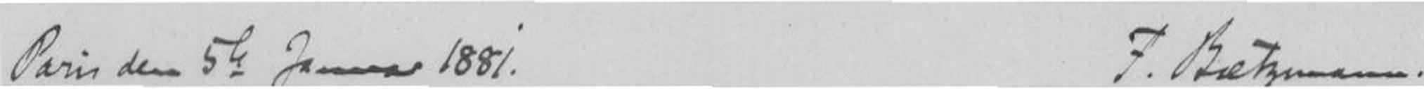Paris den 5te Januar 1881. F. Bætzmann
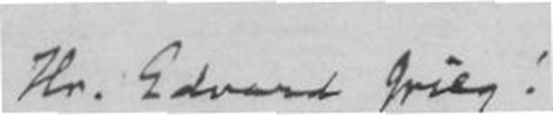Hr Edvard Grieg!
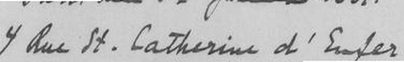4 Rue Catherine d'Enfer
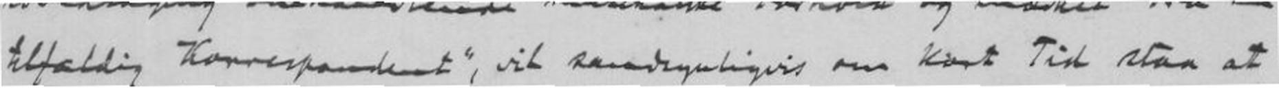tilfældig Korrespondent", vil sandsynligvis om kort Tid staa at
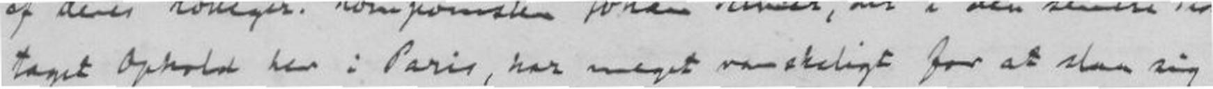taget ophold her i Paris, har meget vanskeligt for at slaa sig
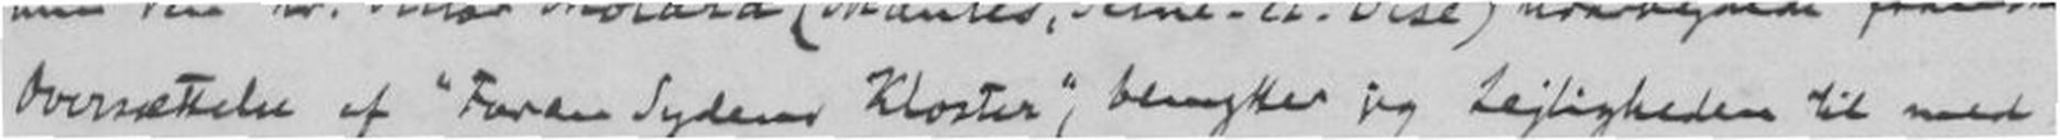Oversættelse af "Foran Sydens Kloster", benytter jeg Lejligheden til med
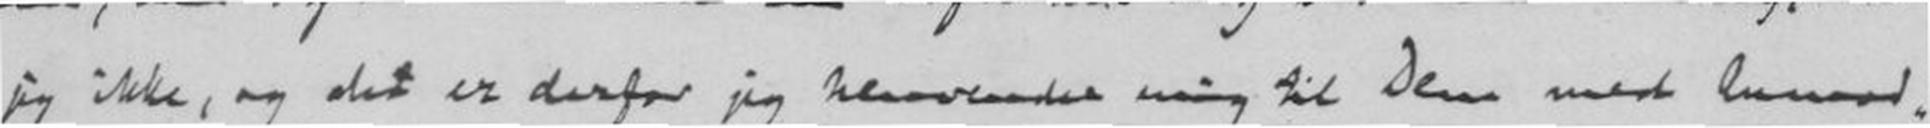jeg ikke, og det er derfor jeg henvender mig til Dem med Anmod-
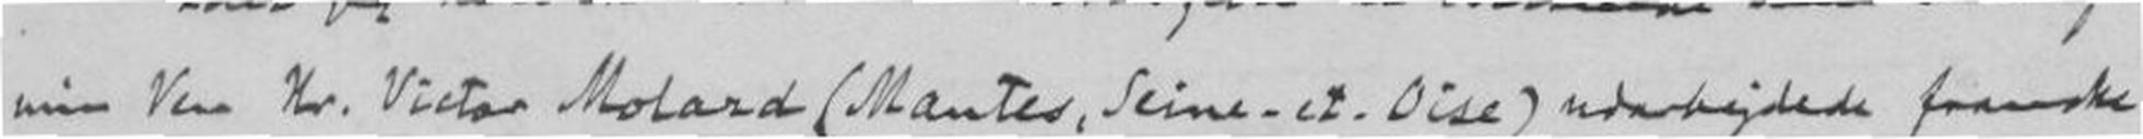min Ven Hr. Victor Molard (Mantes, Seine-et-Dise) udarbejdede franske
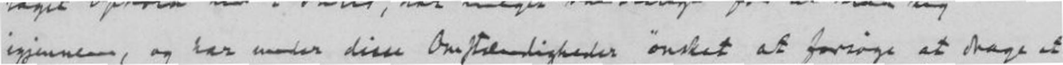igjennem, og har under disse Omstændigheder ønsket at forsøge at drage et
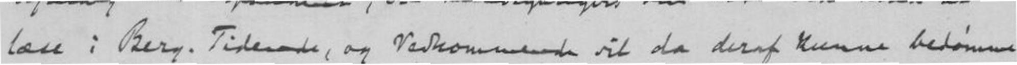læse i Berg. Tidende, og Vedkommende vil da deraf kunne bedømme
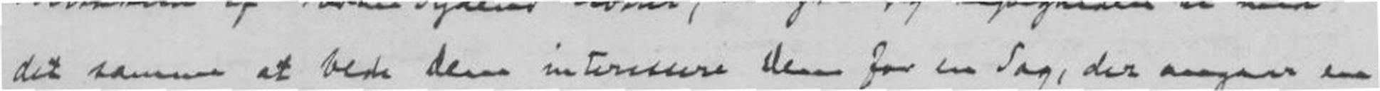det samme at bede dem interessere Dem for en Sag, der angaar en
